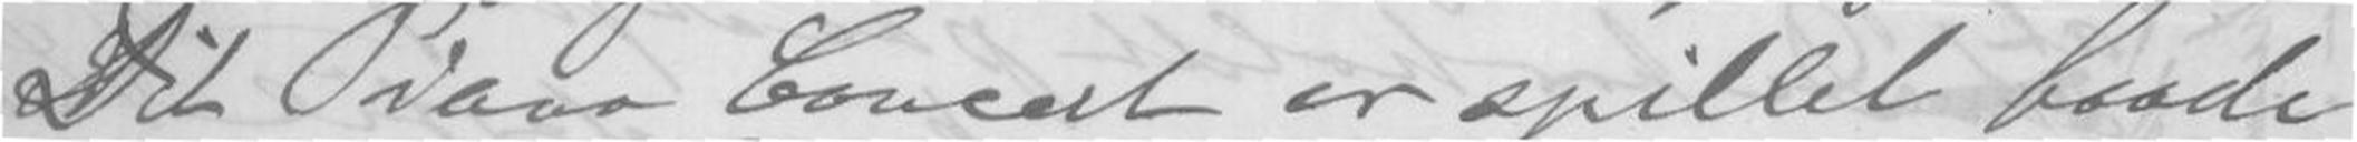Dit Piano Concert er spillet baade
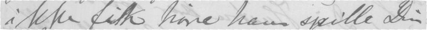ikke fik høre ham spille Din
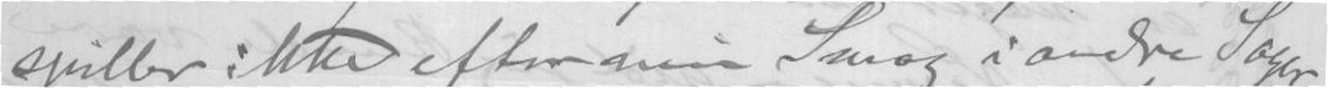spiller ikke efter min Smag i andre Sager
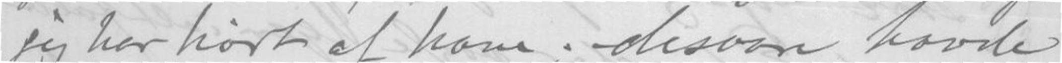jeg har hørt af ham; desvære havde
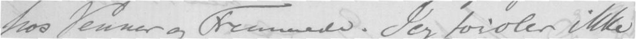hos Venner og Fremmede. Jeg tvivler ikke
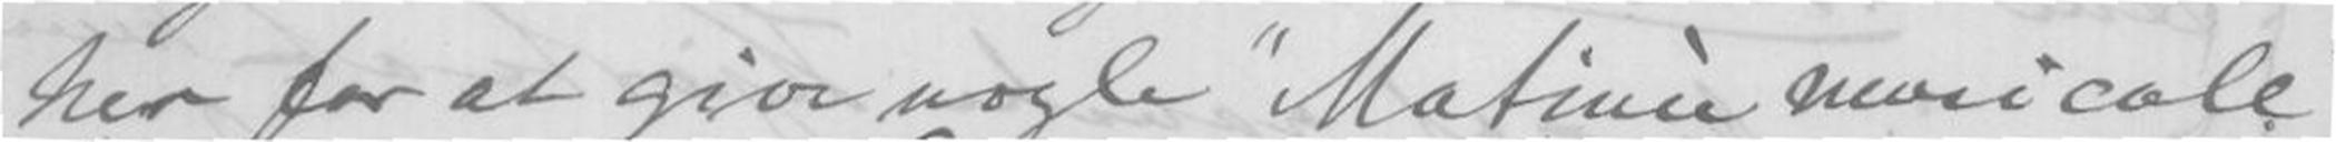her for at give nogle "Matinèe musicale
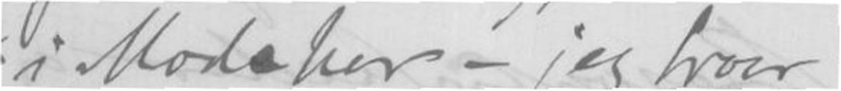i Mode her - jeg tror
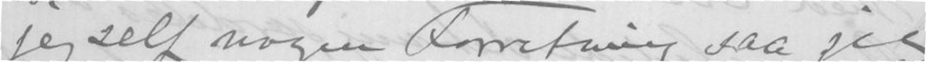jeg self nogen Forretning saa jeg
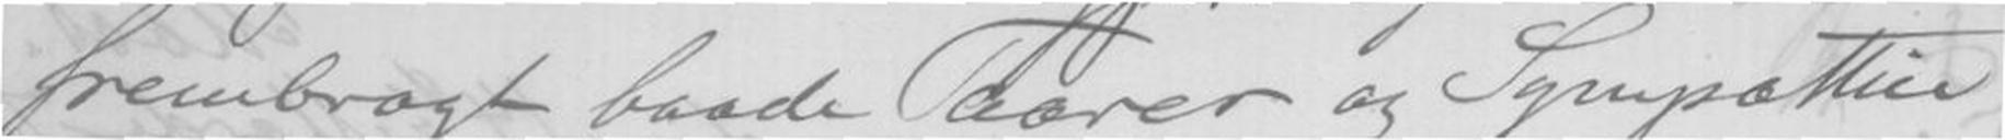frembragt baade Taarer og Sympattier
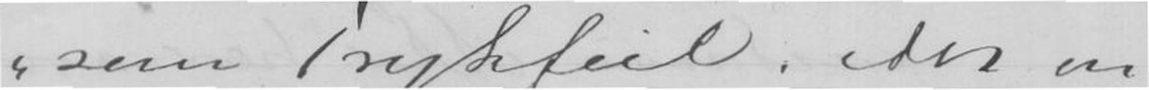som Trykfeil. Der en
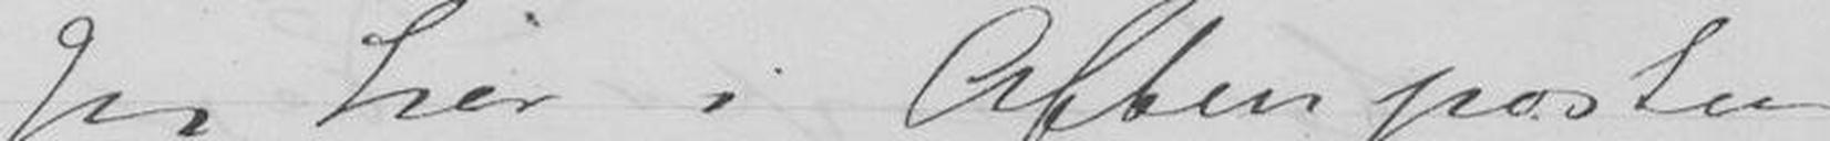Jeg har i Aftenposten
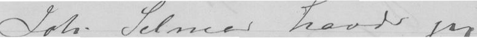Joh. Selmer havde jeg
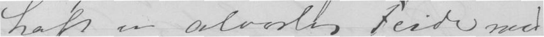haft en alvorlig Feide med
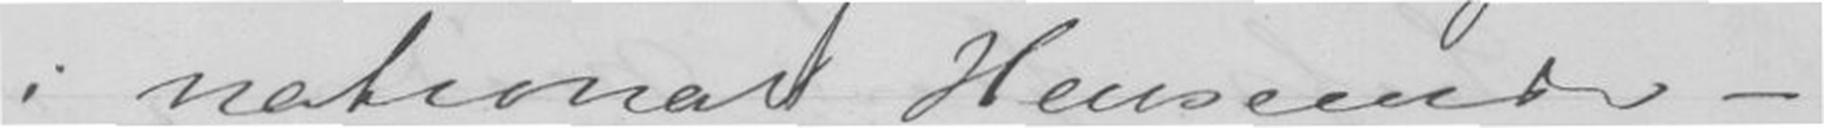i national Henseende -
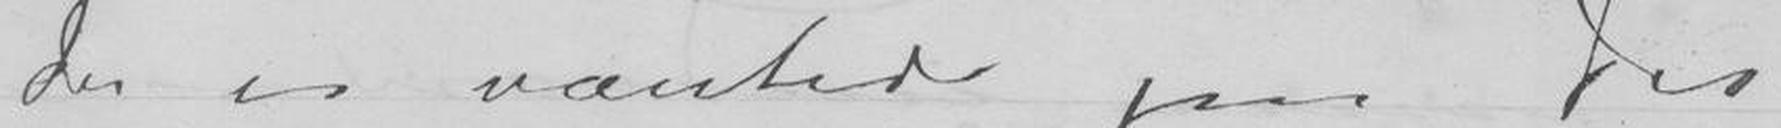da vi væntede paa Dit
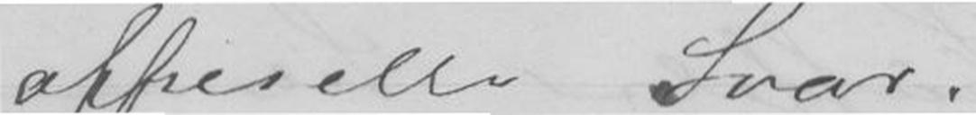officielle Svar.
